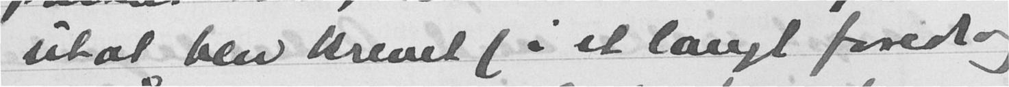utat blir krevet (i et langt foredrag
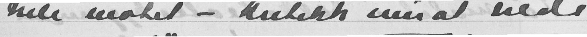hele møtet - kritikk imot vilde
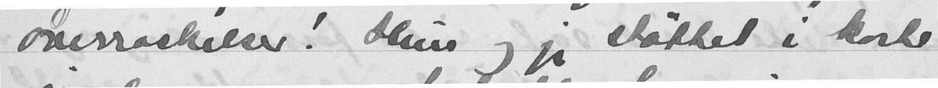overraskelser. Hun og jeg støttet i korte
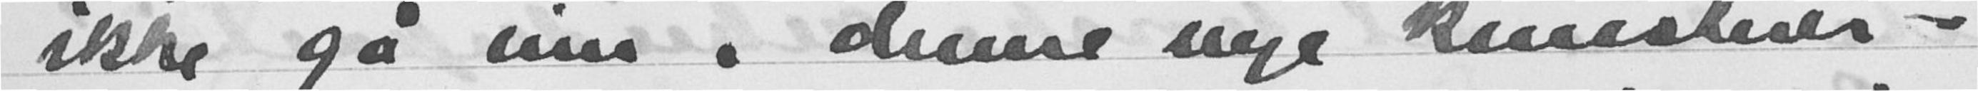ikke gå inn i denne nye kunstner-
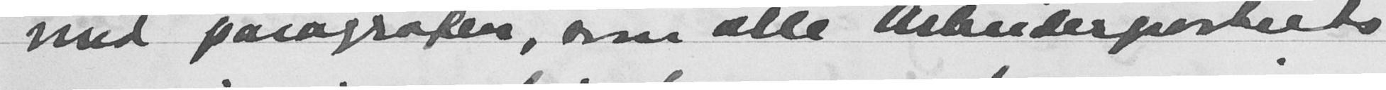med paragrafen, som alle Arbeiderpartiets
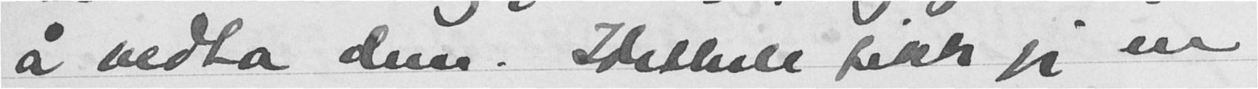å vedta dem. Idethele fikk jeg en
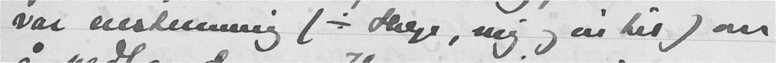var enestemmig (÷ Helge, mig og en til) om
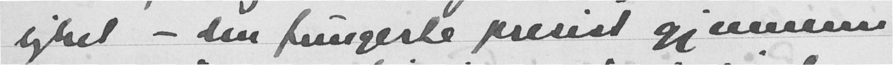lighet - den fungerte presist gjennom
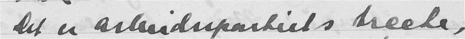Det er arbeidrpartiets brcete,
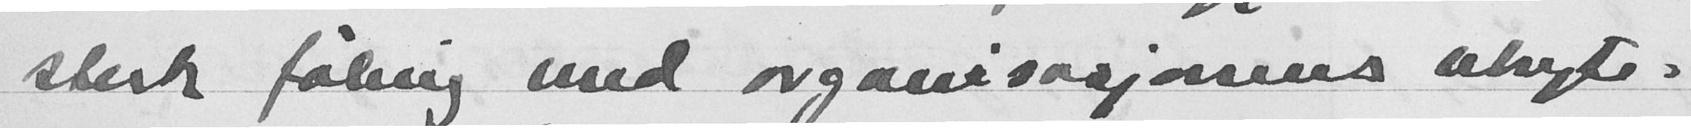sterk føling med organisasjonens ubryte-
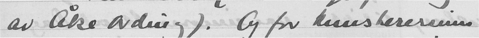av Åke Ording). Og for kunstersinn
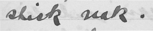stisk nok.
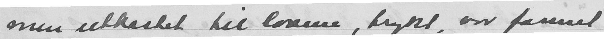men utkastet til lovene, trykt, var formet
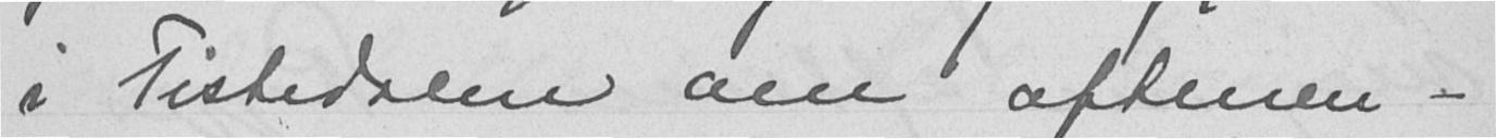i Tistedalen om aftenen -
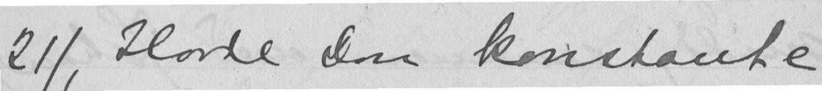21/1. Havde Don konstante
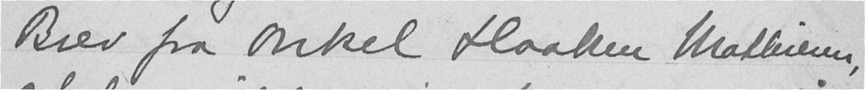Brev fra onkel Haaken Mathiesen,
